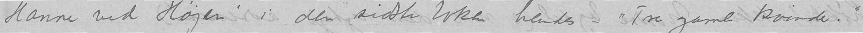«Hanne ved Højen» i den sidste boken hendes «Tre gamle kvinder.»
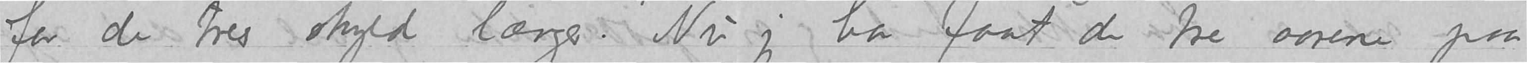for de tres skyld længer. Nu jeg har faat de tre aarene paa Sinsen litt paa
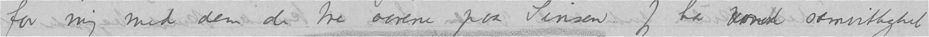for mig med dem de tre aarene paa Sinsen. Jeg har vond samvittighet
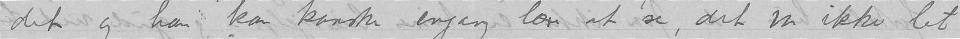det og han kan kanske engang lære at se, det var ikke let
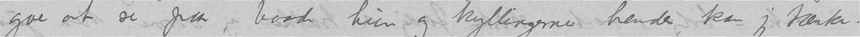goe at se paa, baade hun og kyllingerne hendes, kan jeg tænke.
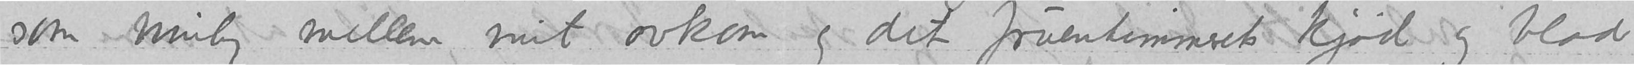som mulig mellem mit avkom og det fruentimmerets kjød
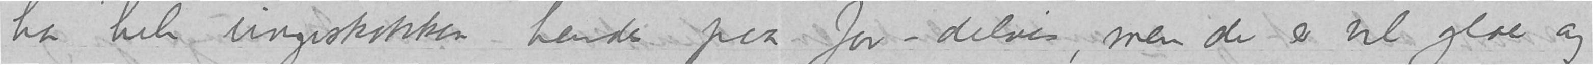ha hele ungeskokken hendes paa for-delvis, men de er vel glae og
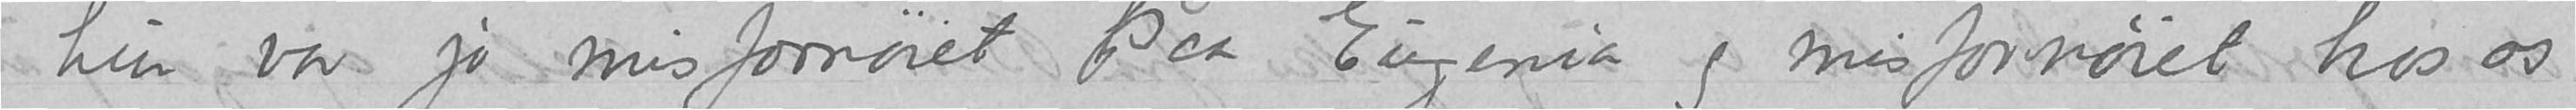-hun var jo misfornøiet paa Eugenia og misfornøiet hos os
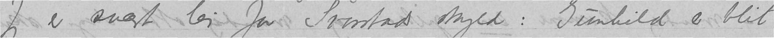Jeg er svært lei for Svarstads skyld: Gunhild er blit
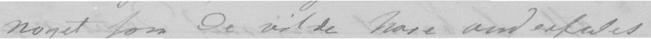noget som De vilde have anderledes
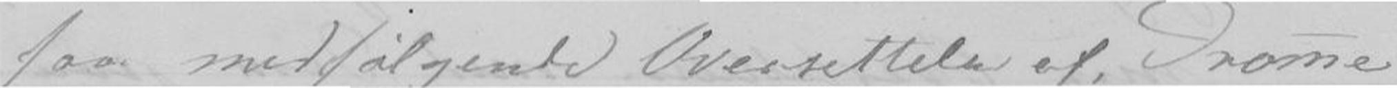faa medfølgende Oversettelse af, Drøme
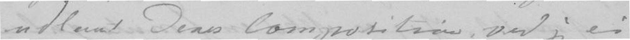udlevert Deres Composition ved jeg ei
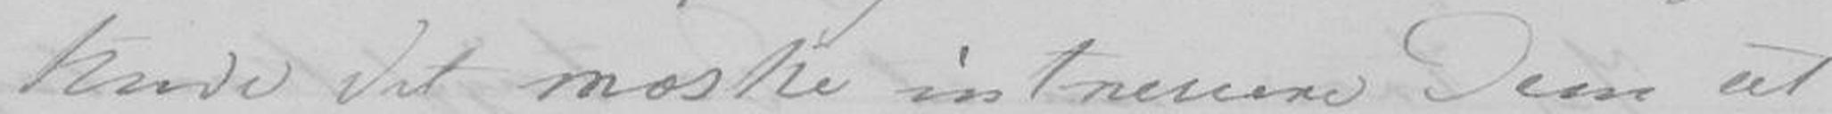kunde det moske intressere Dem at
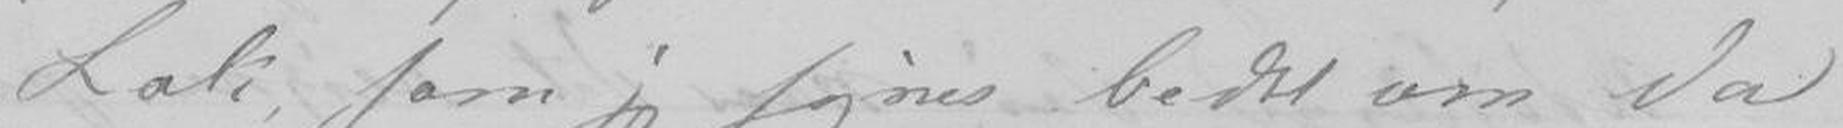Lok, som jeg synes bedst om da
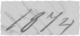1874
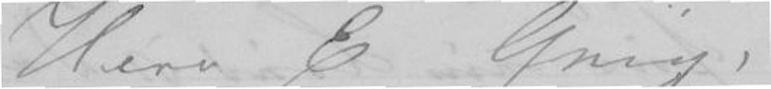Herr E Grieg,
